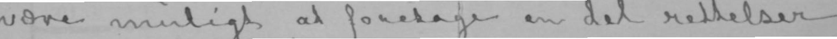være muligt at foretage en del rettelser
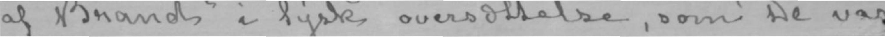af «Brand» i tysk oversættelse, som De var
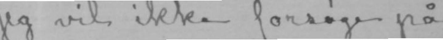Jeg vil ikke forsøge på
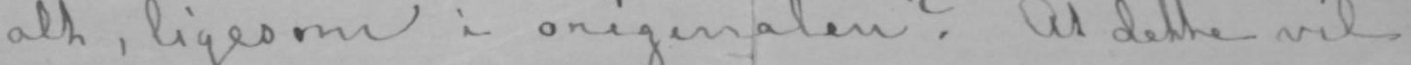alt, ligesom i originalen? At dette vil
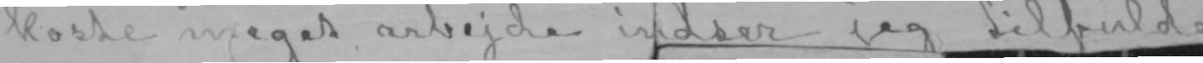koste meget arbejde indser jeg tilfulde
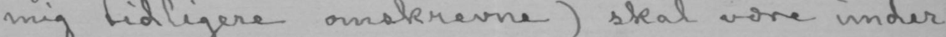mig tidligere omskrevne) skal være under
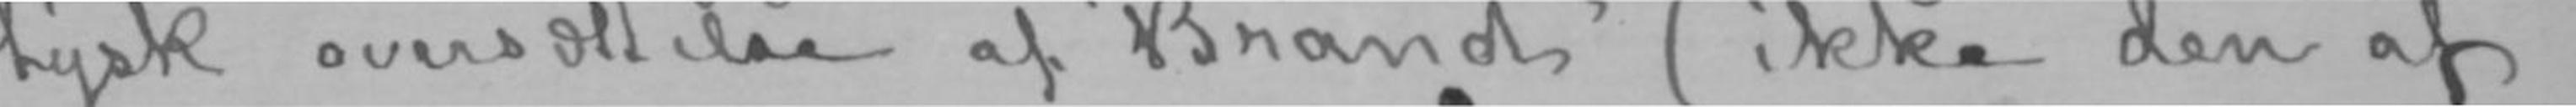tysk oversættelse af «Brand» (ikke den af
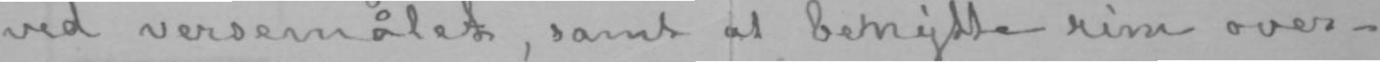ved versemålet, samt at benytte rim over-
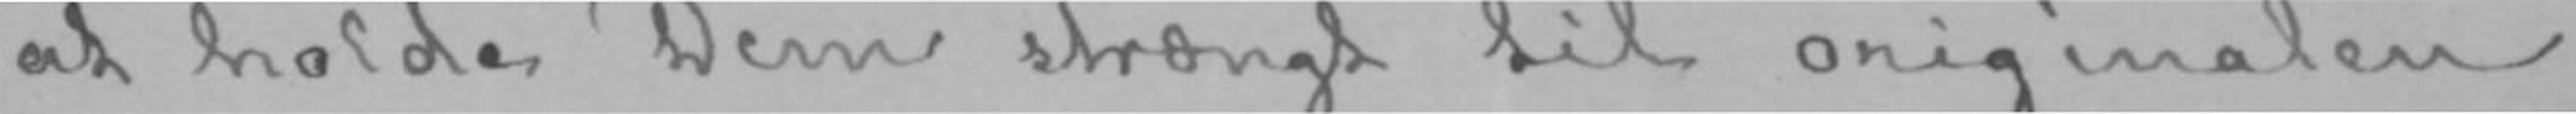at holde Dem strængt til originalen.
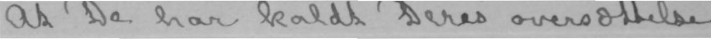At De har kaldt Deres oversættelse
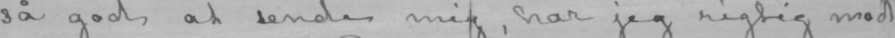så god at sende mig, har jeg rigtig mod-
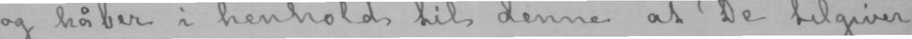og håber i henhold til denne at De tilgiver
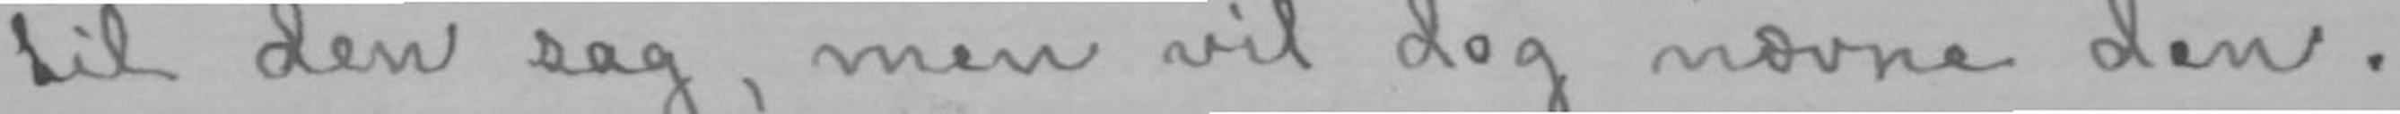til den sag, men vil dog nævne den.
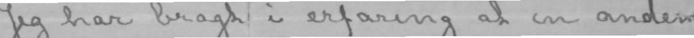Jeg har bragt i erfaring at en anden
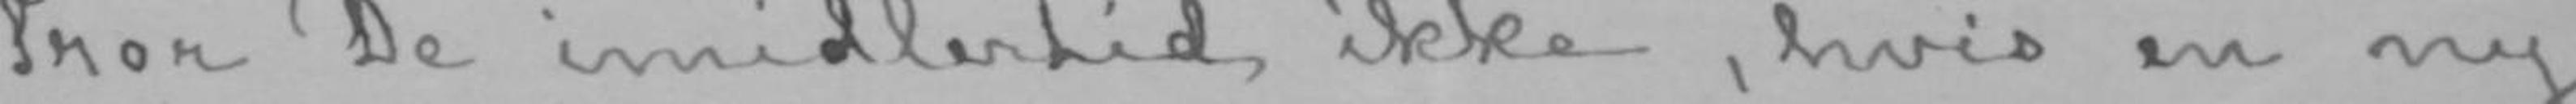Tror De imidlertid ikke, hvis en ny
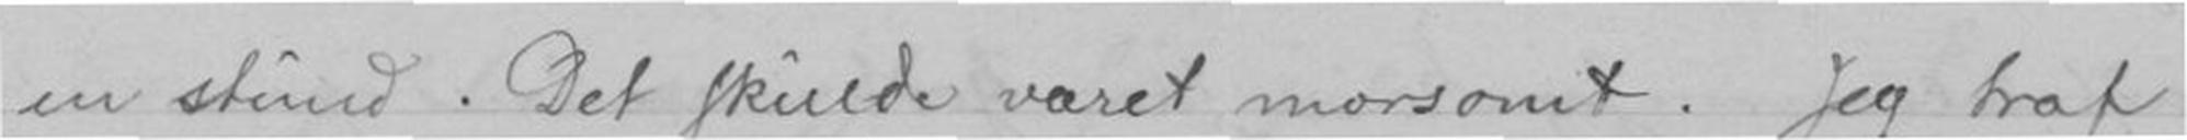en stund. Det skulde været morsomt. Jeg traf
